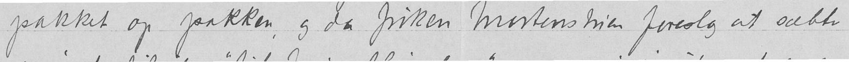pakket op pakken, og da frøken Mortenstuen foreslog at sætte
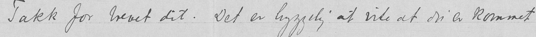Takk for brevet dit. Det er hyggelig at vite at du er kommet
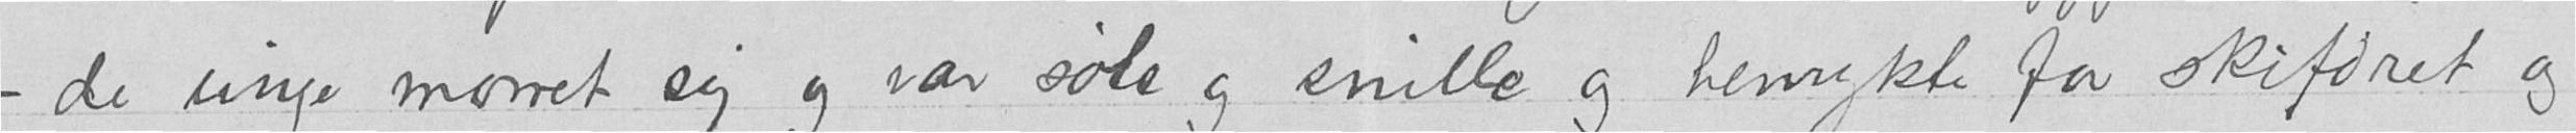– de unge morret sig og var søte og snille og henrykte for skiføret og
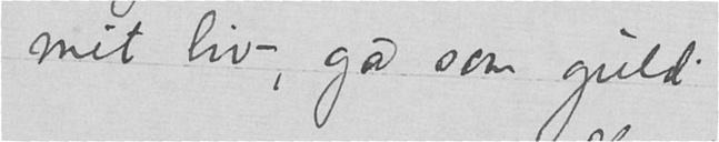mit liv, go som guld.
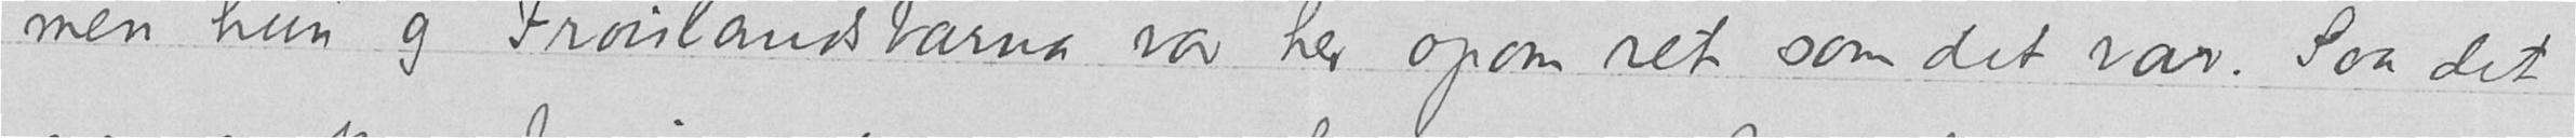men hun og Frøislandsbarna var her opom ret som det var. Saa det
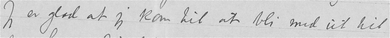Jeg er glad at jeg kom til at bli med ut til
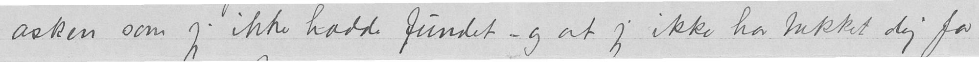asken som jeg ikke hadde fundet – og at jeg ikke har takket dig for
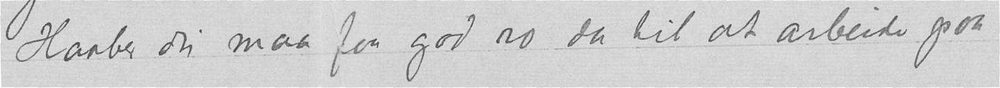Haaber du maa faa god ro da til at arbeide paa
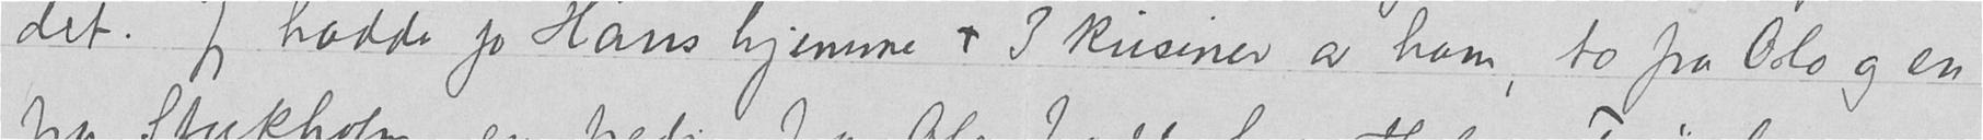det. Jeg hadde jo Hans hjemme + 3 kusiner av ham, to fra Oslo og en
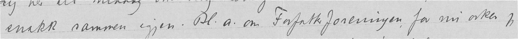snakk sammen igjen. Bl.a. om Forfatterforeningen, for nu orker jeg
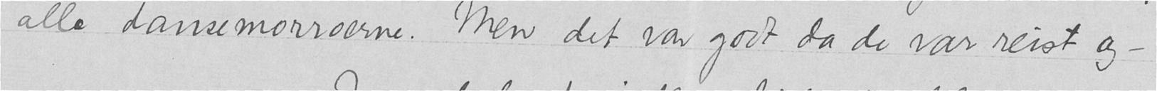alle dansemorroerne. Men det var godt da de var reist og -.
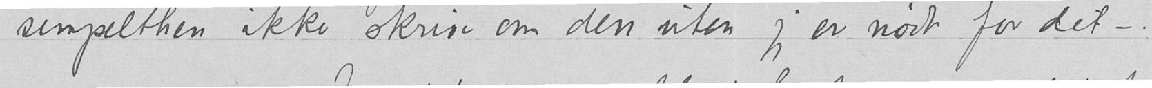simpelthen ikke skrive om den uten jeg er nødt for det –.
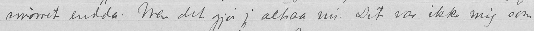smørret endda. Men det gjør jeg altsaa nu. Det var ikke mig som
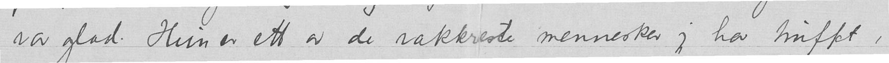var glad. Hun er ett av de vakkreste mennesker jeg har truffet i
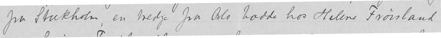fra Stockholm, en tredje fra Oslo bodde hos Helene Frøisland
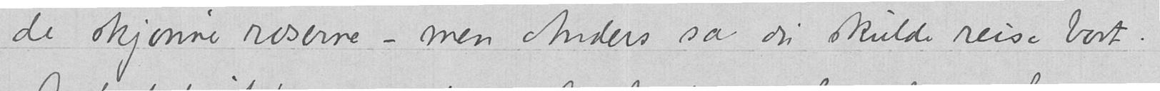de skjønne roserne – men Anders sa du skulde reise bort.
